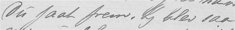Du faat frem. Jeg blev saa
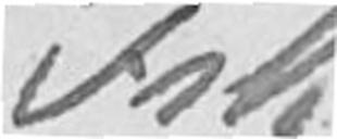Frk
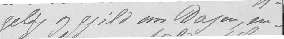gelig og gjild om Dagen, en-
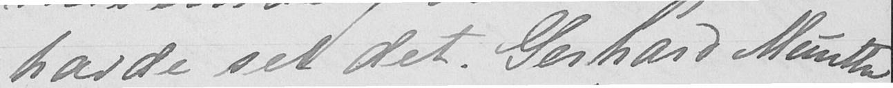havde set det. Gerhard Munthe
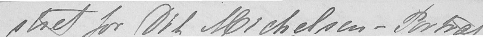stret for Dit Michelsen-Portræt,
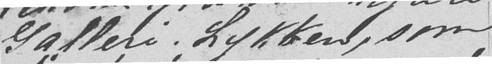Galleri. Lykken, som
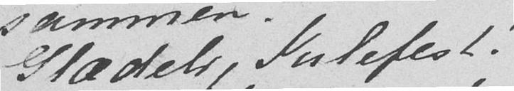Glædelig Julefest!
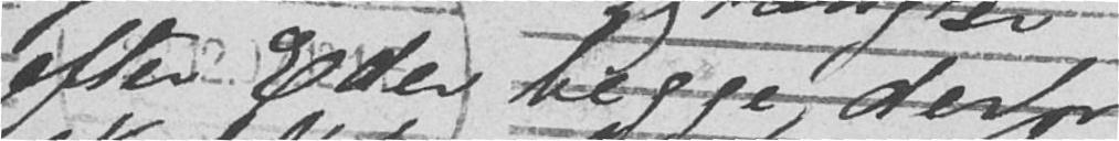efter Eder begge, derfor
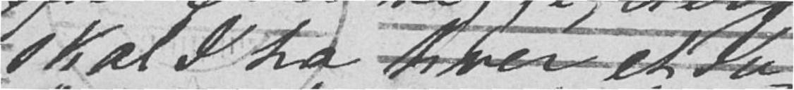skal I ha hver et Ju-
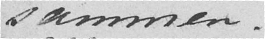sammen.
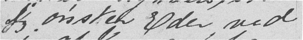Jeg ønsker Eder, ved
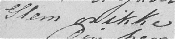Glem os ikke.
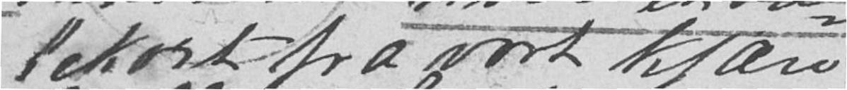lekort fra vort kjære
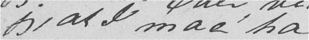jeg, at I maa ha
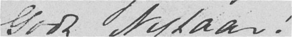Godt Nytaar!
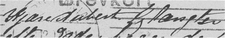Kjære Aubert. Jeg længter
